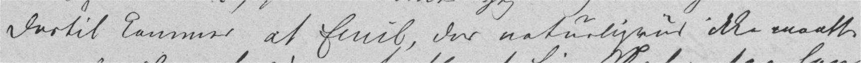Dertil kommer at Emil, der naturligviis ikke maatte
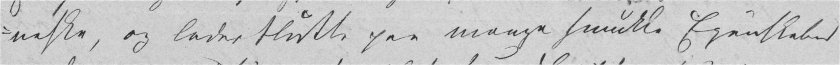neske, og lader slutte paa mange smukke Egenskaber
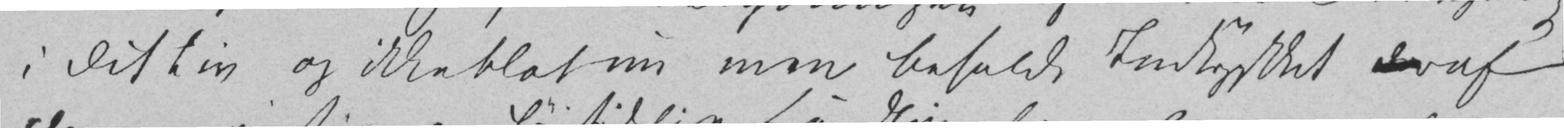i Dit Liv og ikke blot nu men beholde Indtrykket deraf
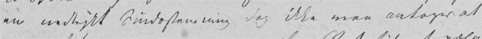en nedtrykt Sindstemning dog ikke maa antages at
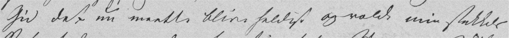Gid det nu maatte blive heldigt og volde min stakkels
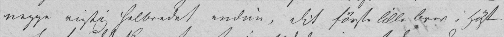neppe rigtig helbredet endnu, Dit første lille Brev i Høst
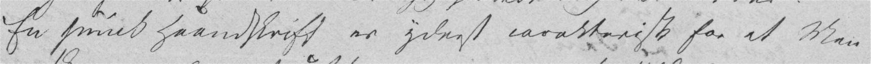En smuk Haandskrift er yderst carakteristisk for et Men-
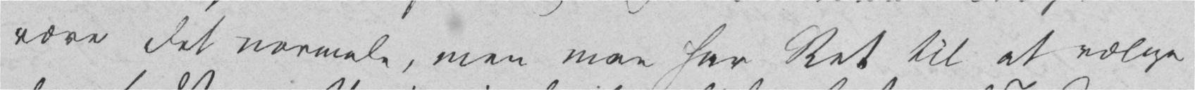være det normale, men man har ret til at vælge
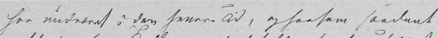har undværet i den senere Tid, og saasom saadant
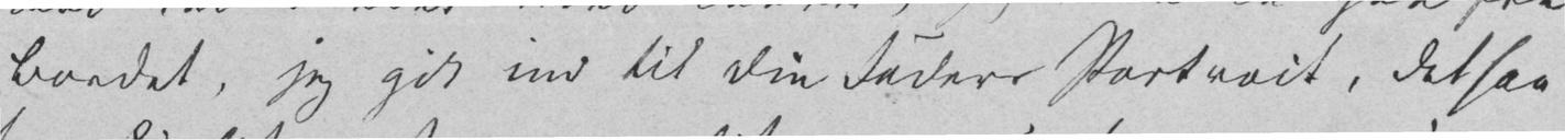Bordet, jeg gik ind til Din Faders Portrait, det saa
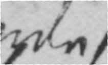ende
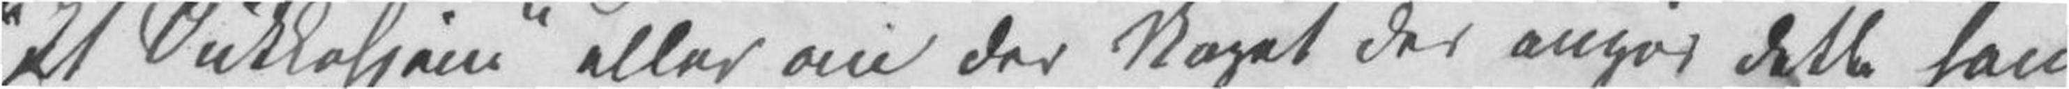«Et Dukkehjem» eller om der Noget der angår dette
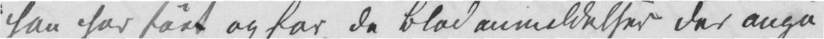han har fået og får de Bladanmeldelser der angå
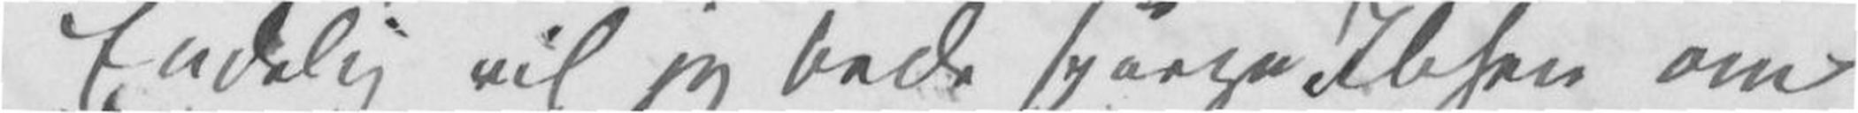Endelig vil jeg bede spørge Ibsen om
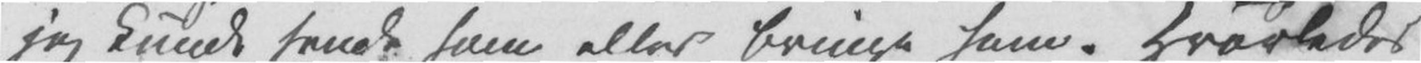som jeg kunde sende ham eller bringe ham. Hvorledes
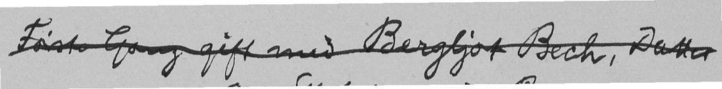Føste Gang gift med Bergljot Bech, Datter
